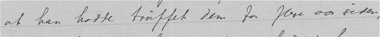at han hadde truffet Dem for flere aar siden,
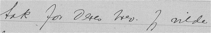tak for Deres brev. Jeg vilde
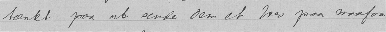tænkt paa at sende Dem et brev paa maafaa
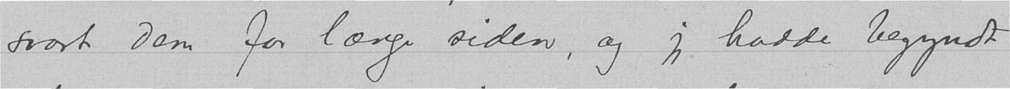svart Dem for længe siden, og jeg hadde begyndt
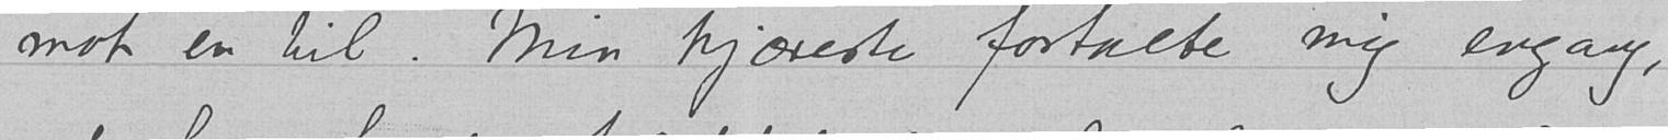mot en til. Min kjæreste fortalte mig engang,
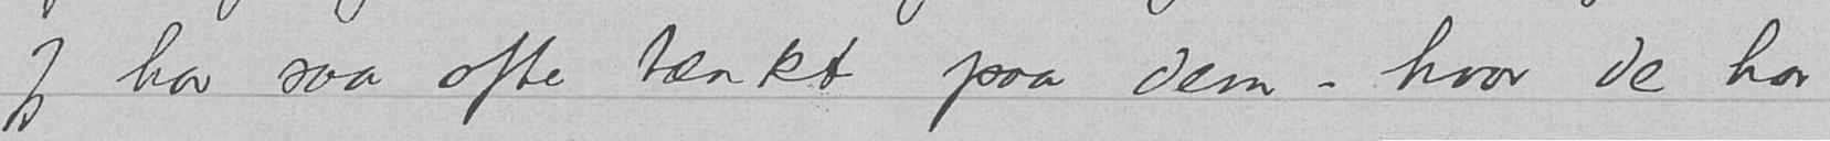Jeg har saa ofte tænkt paa Dem – hvor De har
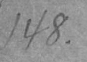148.
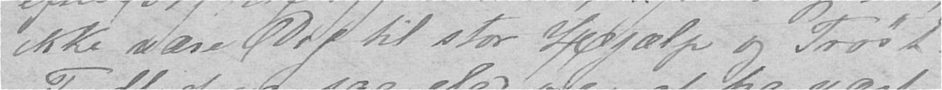ikke være Dig til stor Hjælp og Trøst.
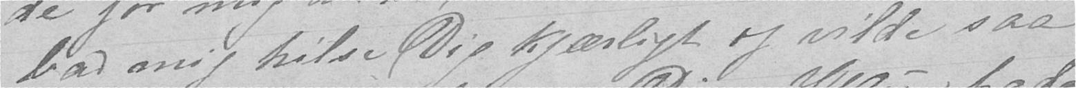bad mig hilse Dig kjærligt og vilde saa
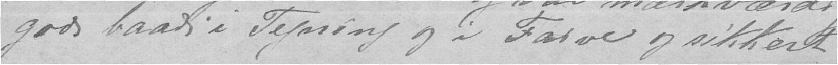gode baade i Tegning og i Farver og sikkert
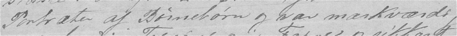Portræter af Børnebørn og var mærkværds
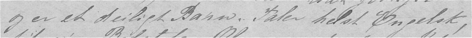og er et deiligt Barn. Taler helst Engelsk,
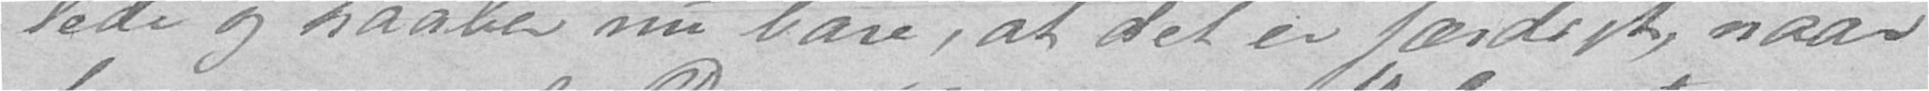lede og haaber nu bare, at det er færdigt, naar
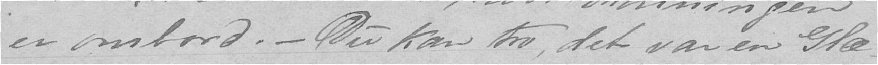en ombord. - Du kan tro, det var en Glæ-
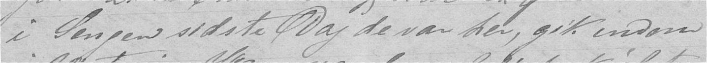i Sengen sidste Dag de var her, gik indom
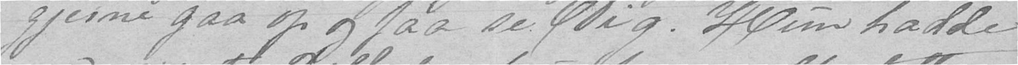gjerne gaa op og faa se Dig. Hun hadde
